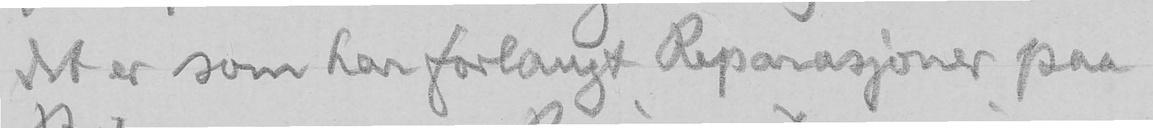det er som har forlangt Reparasjoner paa
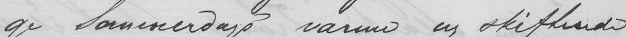ge Sommerdags varm og skiftende
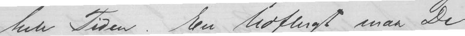hele Tiden. En Udflugt maa De
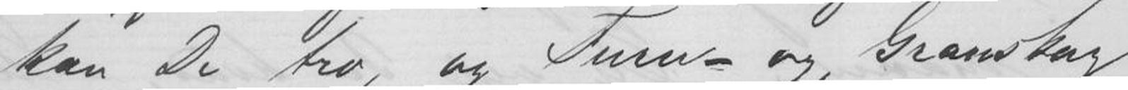kan De tro, og Furu- og Granskog
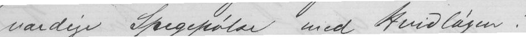værdige Spegepølse med Hvidløgen!
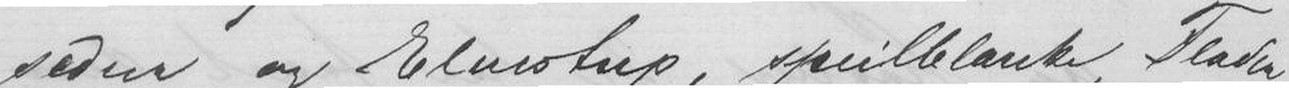sedur og Elvestup, speilblank Flader,
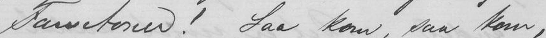Farvetoner! Saa kom, saa kom, -
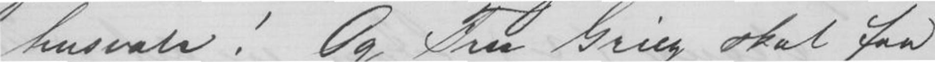husvale! Og Fru Grieg skal faa
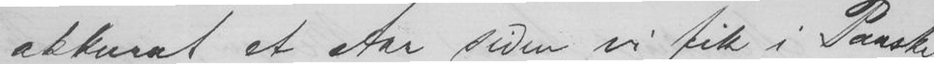akkurat et Aar siden vi fik i Paaske-
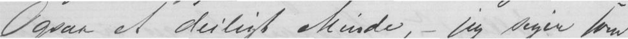Ogsaa et deiligt Minde, - jeg siger som
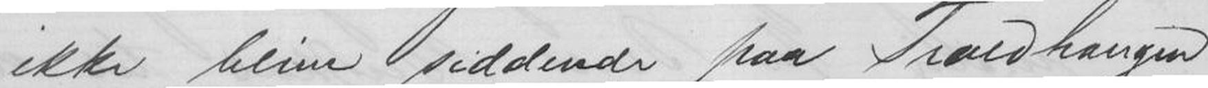ikke blive siddende paa Troldhaugen
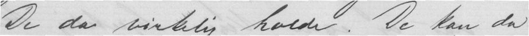De da verkelig holde. De kan da
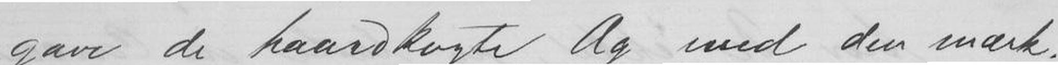gave de haardkogte Æg med den mærk-
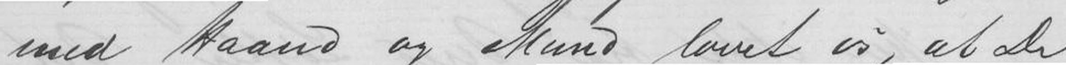med Haand og Mund lovet os, at De
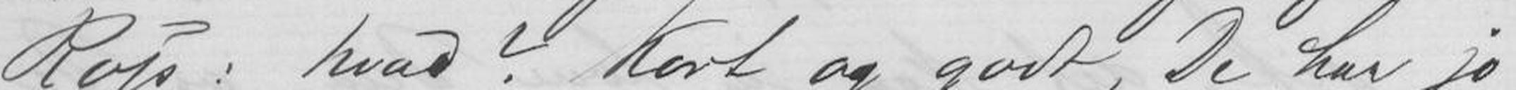Ross: hvad? Kort og godt, De har jo
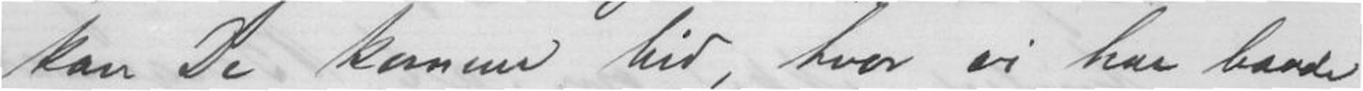kan De komme hid, hvor vi har baade
